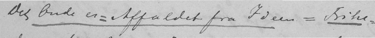Det Onde er = Affaldet fra Ideen = Frihe-
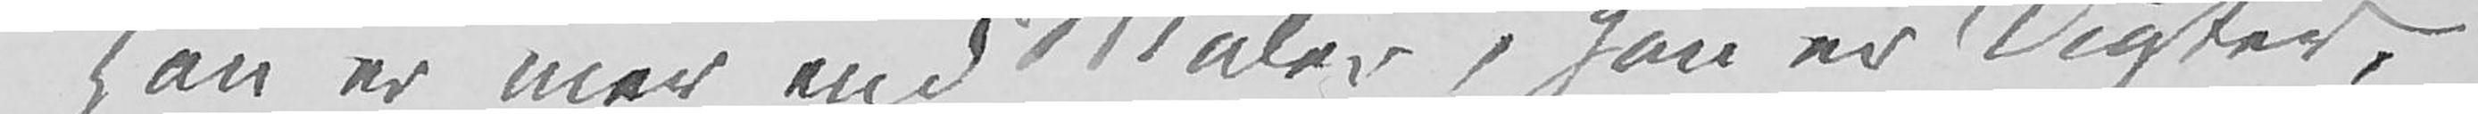Han er mer end Maler, han er Digter,
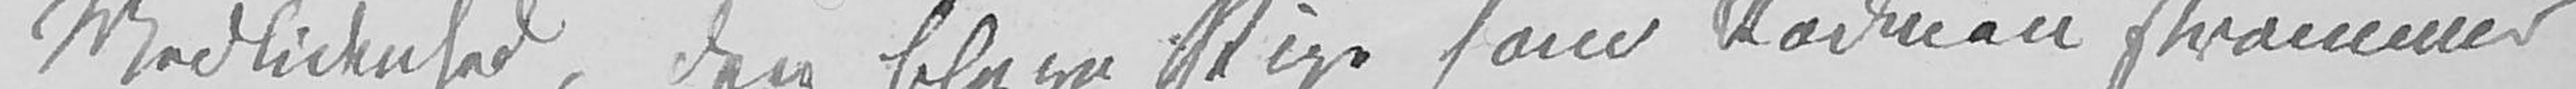Medlidenhed, den blege Pige som Rødmen strømmer
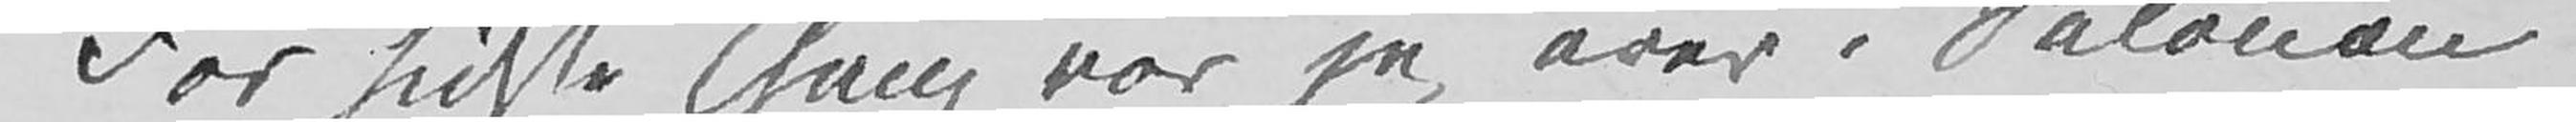For sidste Gang var jeg over i Salonen
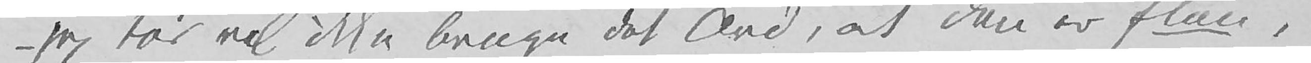– jeg tør vel ikke bruge det Ord, at den er flau,
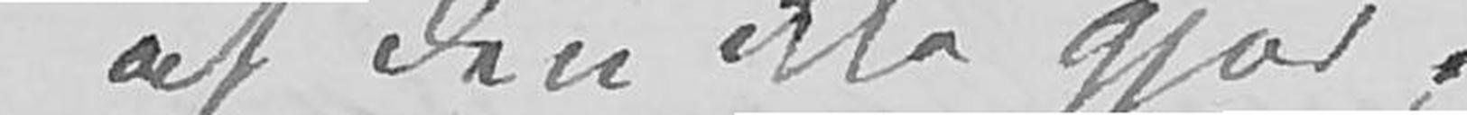at den ikke gjør.
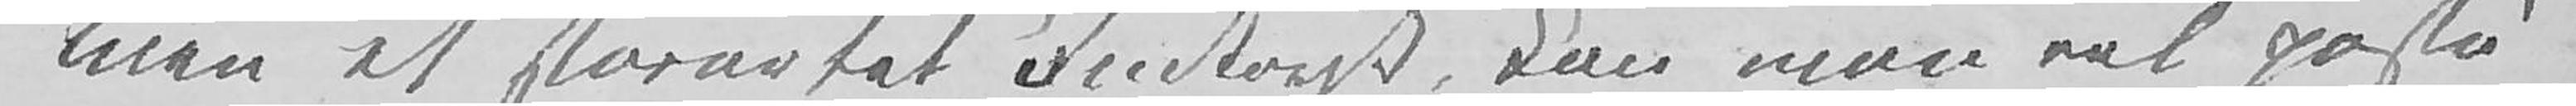men et storartet Indtryk, kan man vel påstå
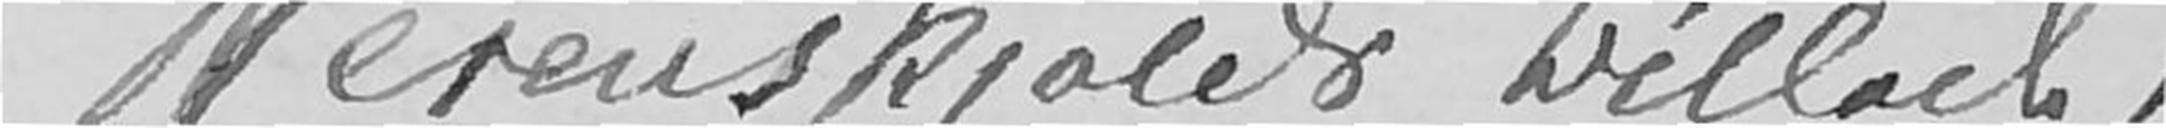Werenskjolds Billede,
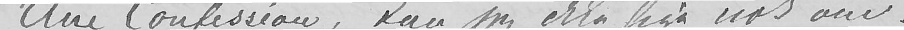Une Confession, kan jeg ikke sige nok om.
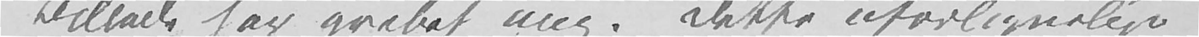Billede har grebet mig. Dette uforlignelige
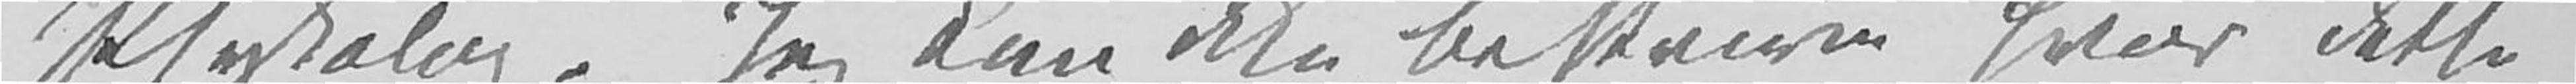Psykolog. Jeg kan ikke beskrive hvor dette
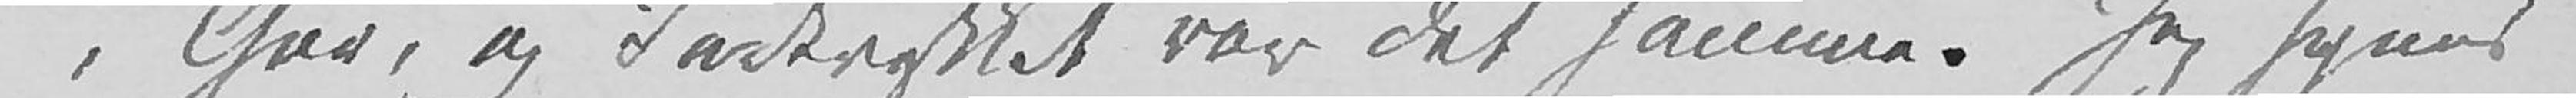i Går, og Indtrykket var det samme. Jeg synes
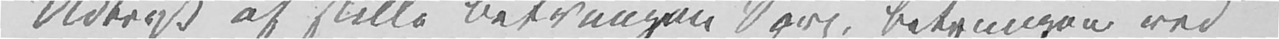Udtryk af stille betvungen Sorg, betvungen ved
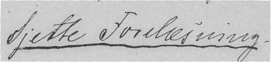Sjette Forelæsning
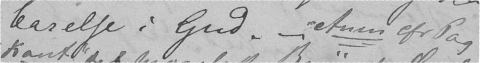barelse i Gud. – Abn cfr Pag
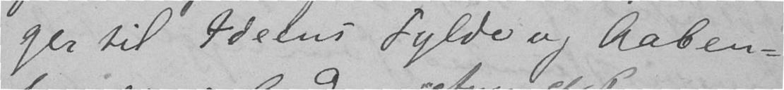ger til Ideens Fylde og Aaben-
